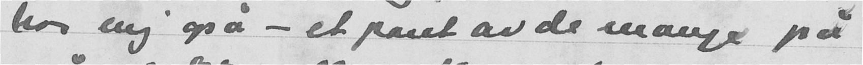hos mig opå - et pant av de mange på
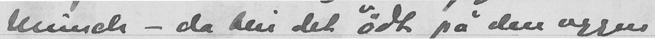Munch - da blir det ødt pa den veggen
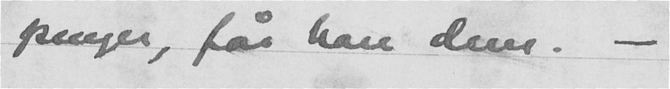penger, får han dem. -
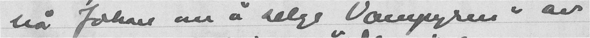nå Johan om å selge "Vampyren" av
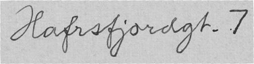Hafrsfjordgt. 7
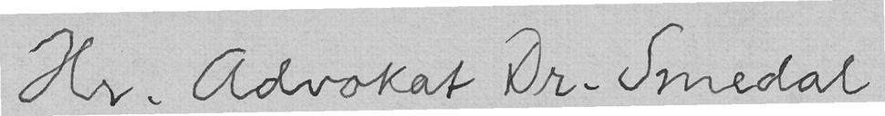Hr. Advokat Dr. Smedal
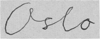Oslo
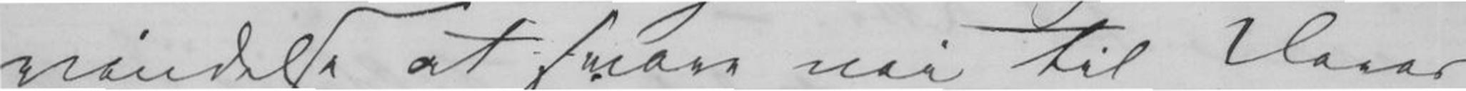mindelig at svare nei til Deres
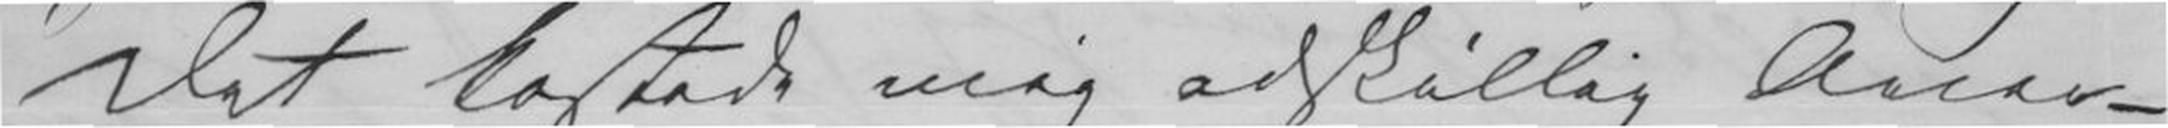Det kostede mig adskillig Over-
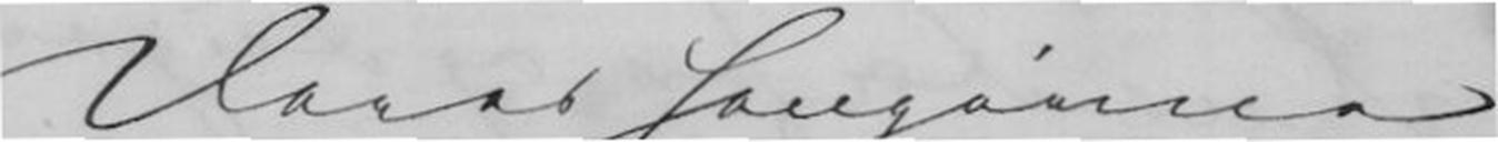Deres hengivne
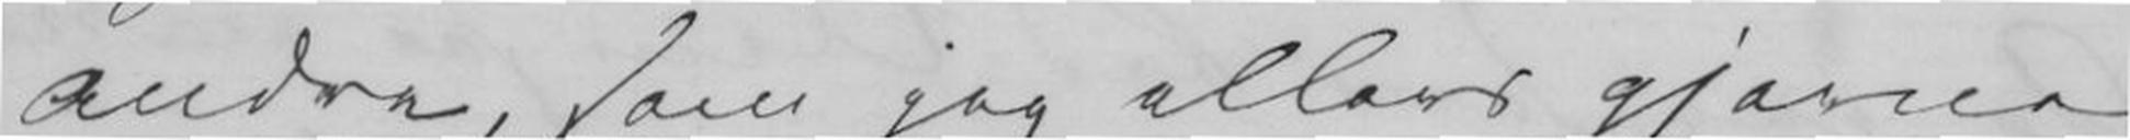andre, som jeg ellers gjerne
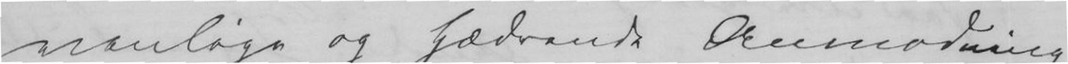venlige og hædrende Anmodning
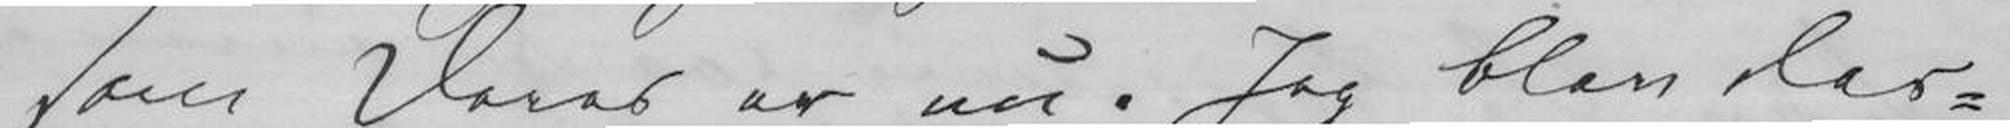som Deres er nå. Jeg blev des-
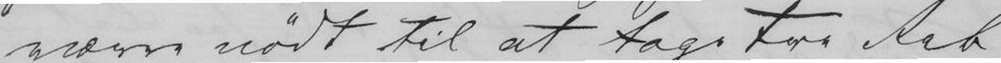værre nødt til at tage tre Reb
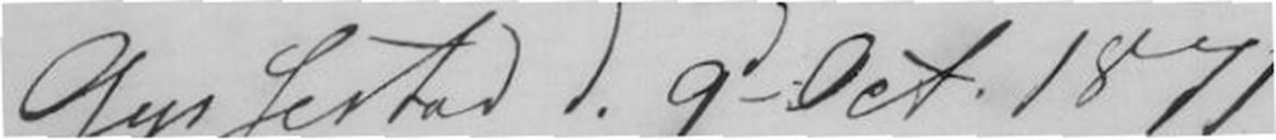Gyssestad d. 9d Oct. 1871.
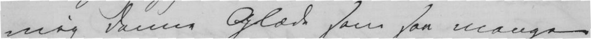mig denne Glæde som saa mange
